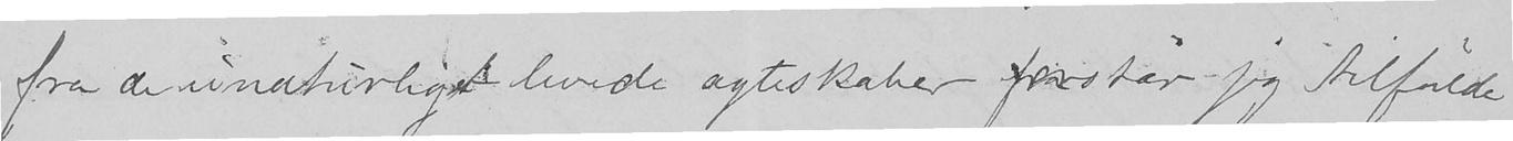fra de unaturligt levede ægteskaber forstår jeg tilfulde.
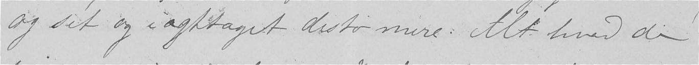og sét og iagttaget desto mere. Alt hvad de
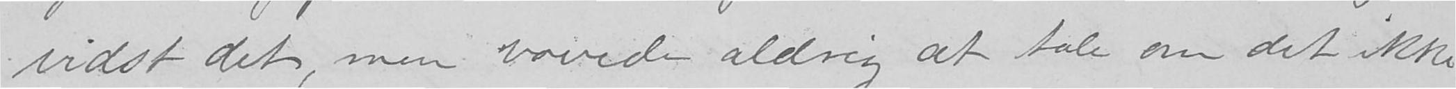vidst det, men vovede aldrig at tale om det ikke
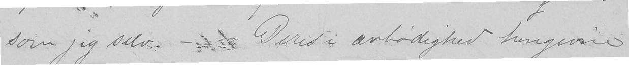som jeg selv. - Deres i ærbødighed hengivne
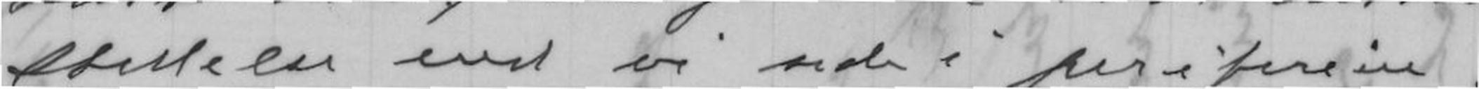stillelse end vi ude i periferien.
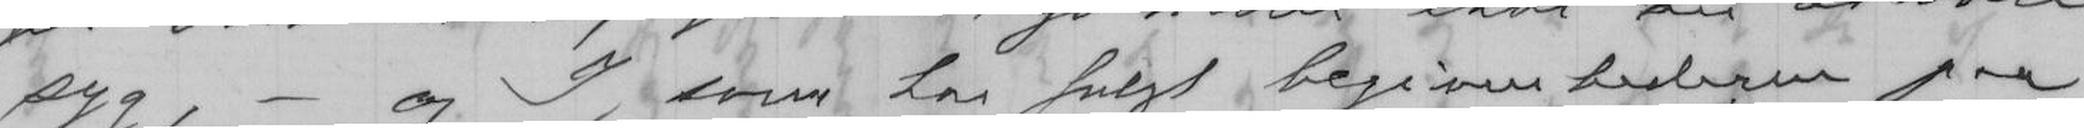syg, - og I, som har fulgt begivenhederne paa
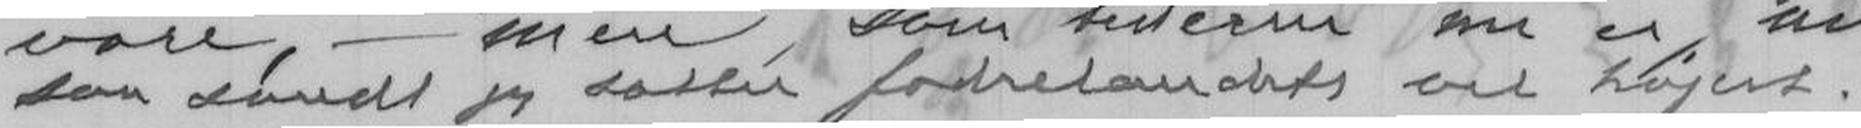saa sandt jeg sætter fædrelandets vel højest.
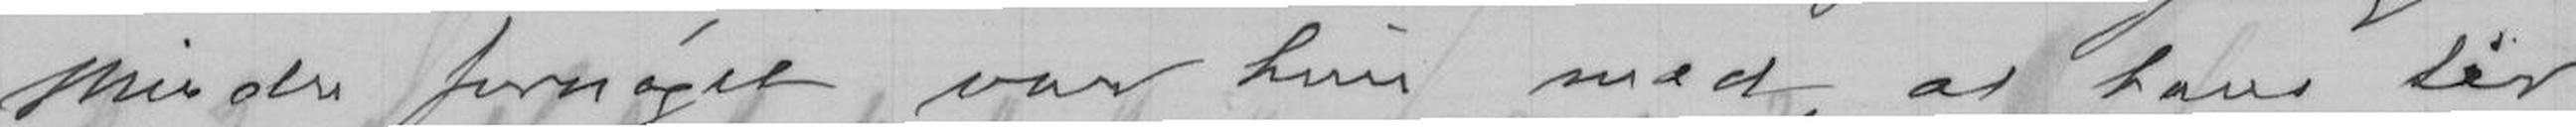Mindre fornøjet var hun med, at hans tid
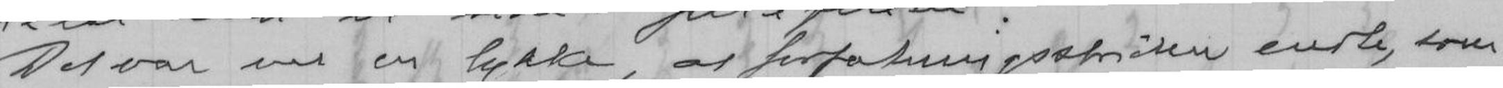Det var en lykke, at forfatningsstriden endte, som
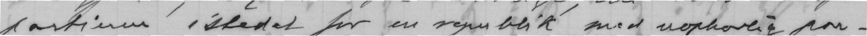partierne istedet for en republik med uophavlig par-
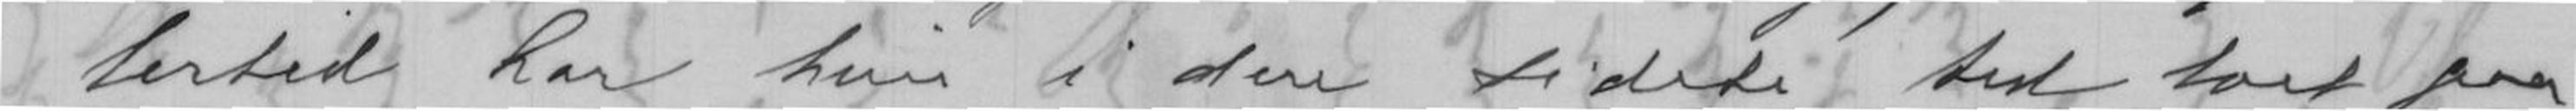lertid har hun i den sidste tid læst paa
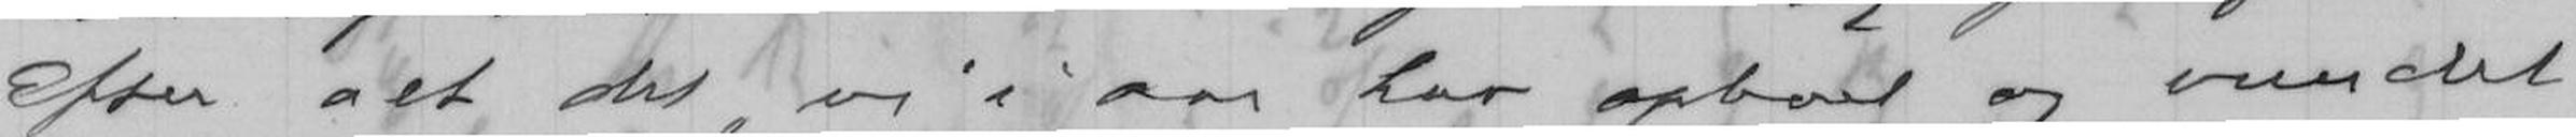Efter alt det, vi i aar har oplevet og vundet
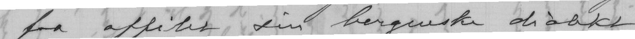faa affilet sin bergenske dialekt
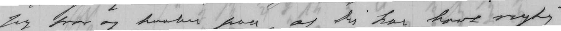Jeg tror og haaber paa, at Du har havt rigtig
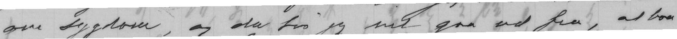om sygdom, og da tør jeg vel gaa ud fra, at baa-
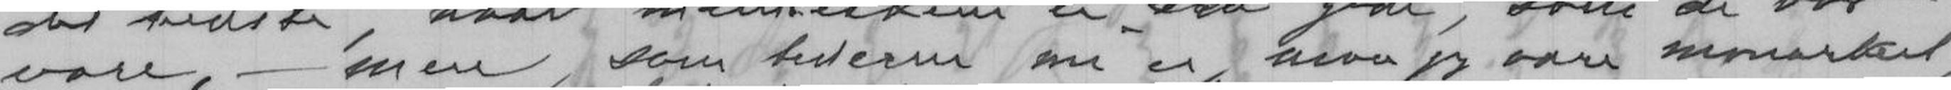være, - men som tiderne nu er, maa jeg være monarkiet,
